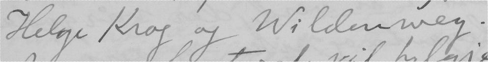Helge Krog og Wildenwey.
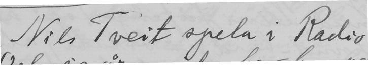Nils Tveit spela i Radio
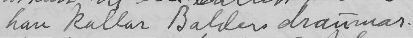han kallar Balders draumar.
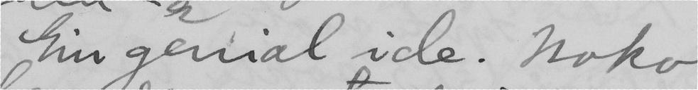Ein genial ide. Noko
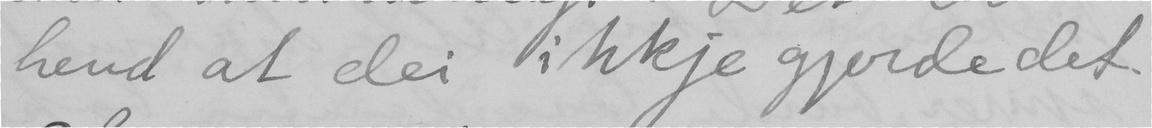hend at dei ikkje gjorde det.
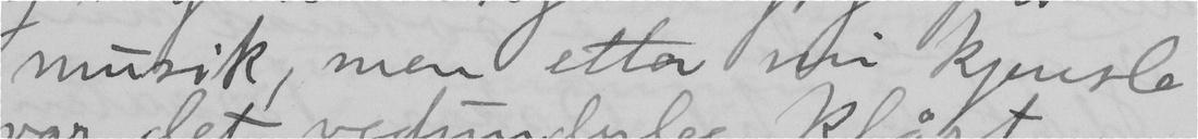musik, men etter mi kjensle
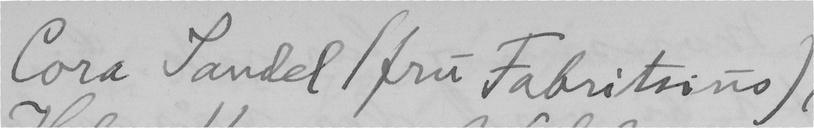Cora Sandel (fru Fabritsius),
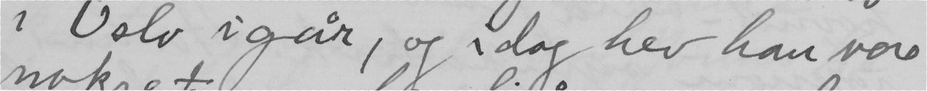i Oslo igår, og idag hev han vore
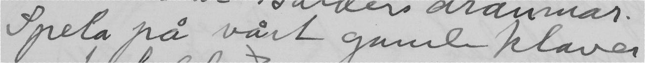Spela på vårt gamle klaver
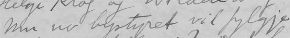Um no bystyret vil fylgje
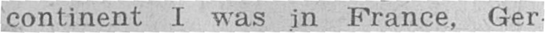continent I was in France, Ger-
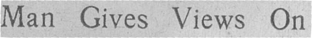Man Gives Views On
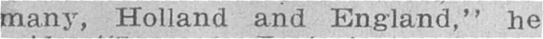many, Holland and England," he
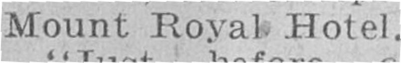Mount Royal Hotel.
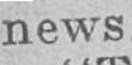news.
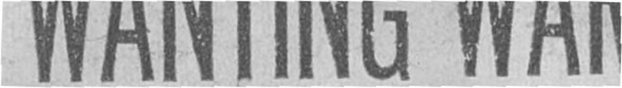NOT WANTING WAR
